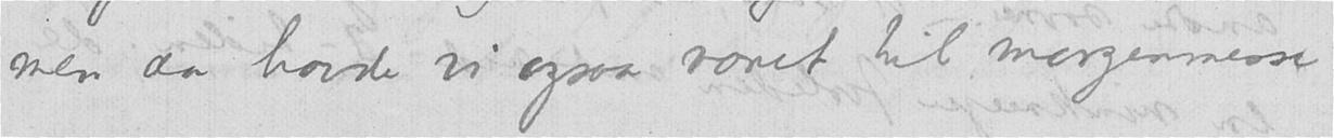men da havde vi ogsaa været til morgenmesse
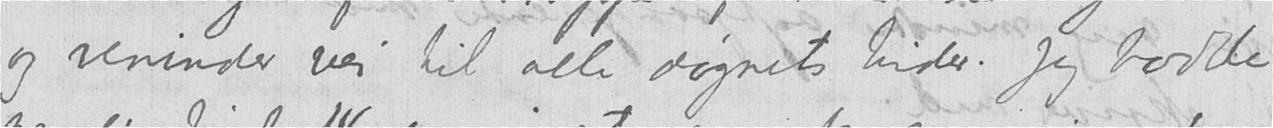og veninder vei til alle døgnets tider. Jeg bodde
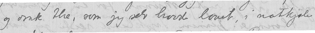og drak the, som jeg selv havde lavet, i natkjole
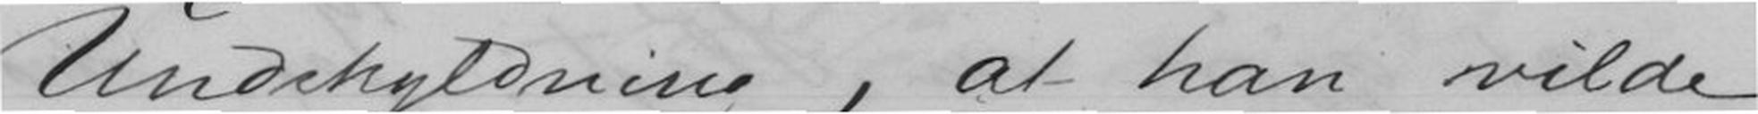Undskyldning, at han vilde
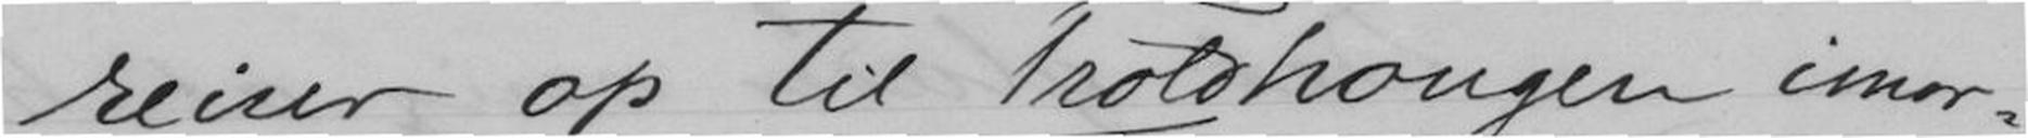reiser op til Troldhaugen imor-
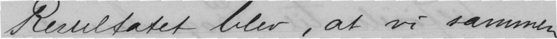Resultatet blev, at vi sammen
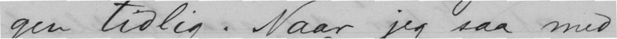gen tidlig. Naar jeg saa med
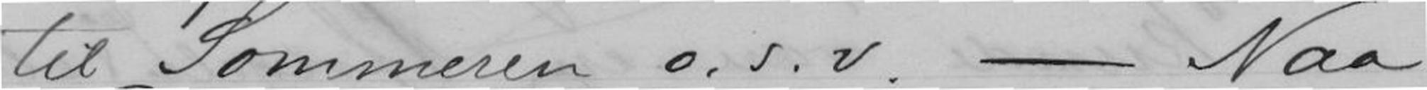til Sommeren o.s.v. _ Naa
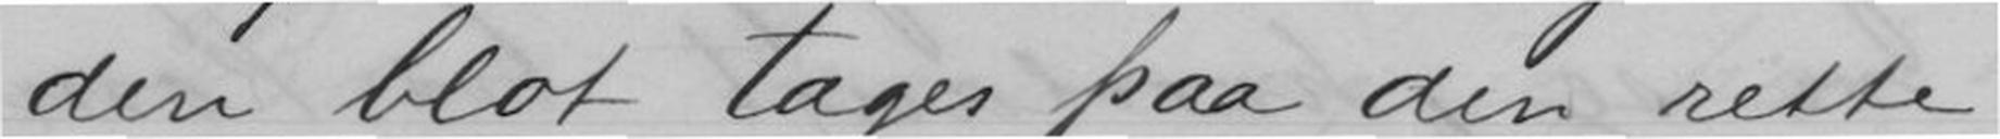den blot tages paa den rette
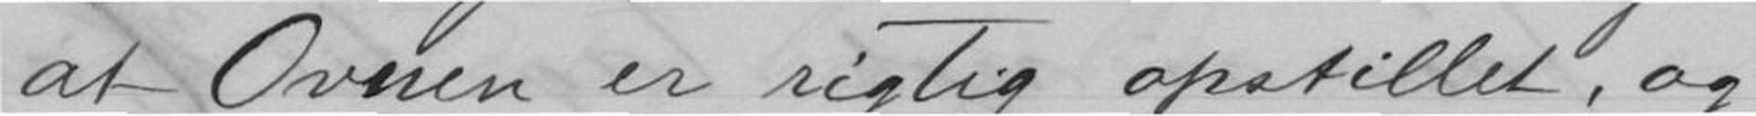at Ovnen er rigtig opstillet, og
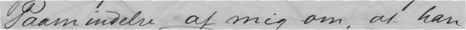Paamindelse af mig om, at han
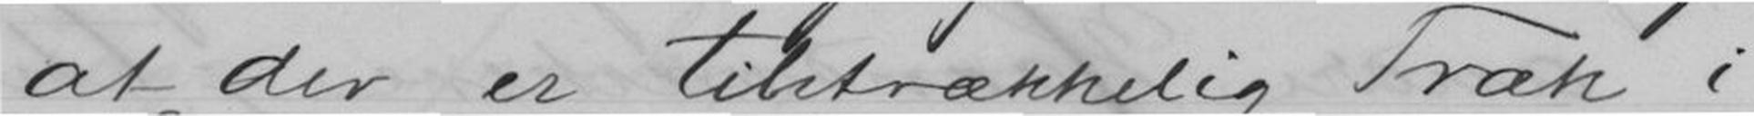at der er tilstrekkelig Træk i
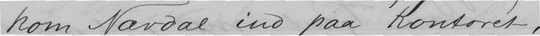kom Nævdal ind paa Kontoret,
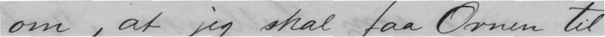om, at jeg skal faa Ovnen til
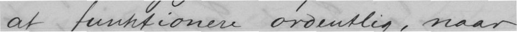at funktionere ordentlig, naar
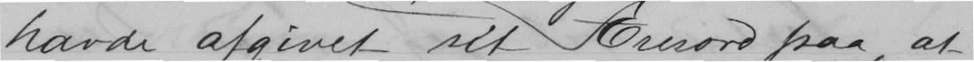havde afgivet sit Æresord paa, at
